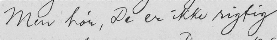Men hør, De er ikke rigtig
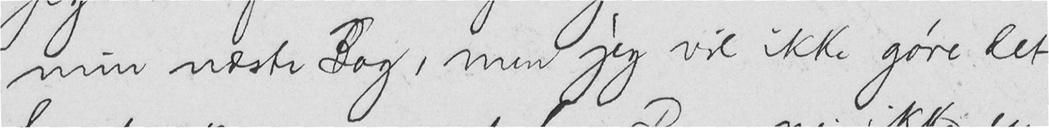min næste Bog, men jeg vil ikke gøre det
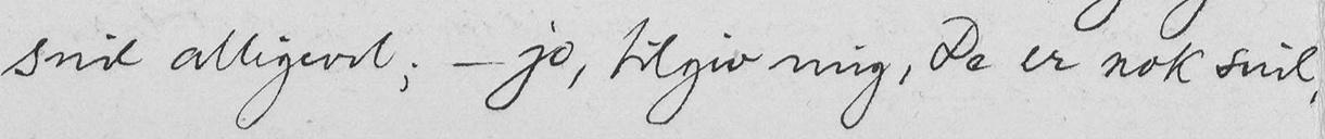snil alligevel; - jo, tilgiv mig, De er nok snil,
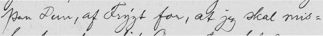paa Dem, af Frygt for, at jeg skal mis-
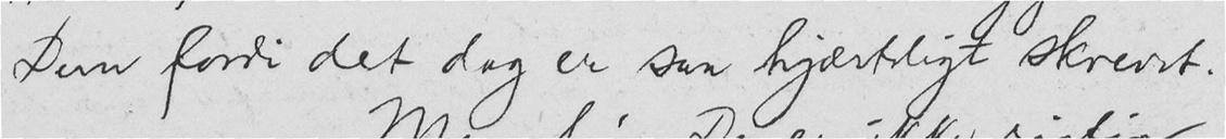Dem fordi det dog er saa hjærteligt skrevet.
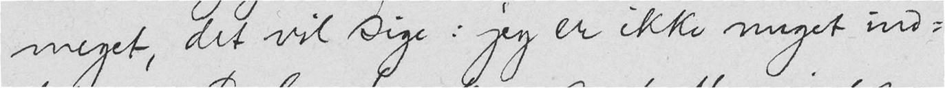meget, det vil sige: jeg er ikke meget ind-
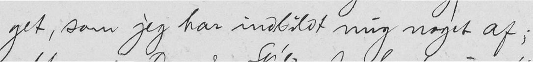get, som jeg har indbildt mig noget af;
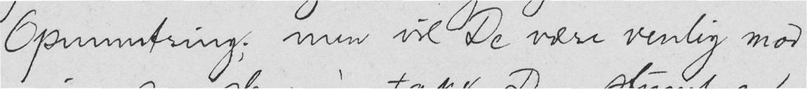Opmuntring; men vil De være venlig mod
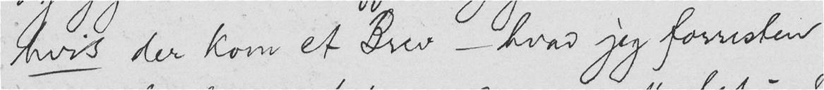hvis der kom et Brev - hvad jeg forresten
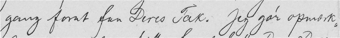gang forat faa Deres Tak. Jeg gør opmærk-
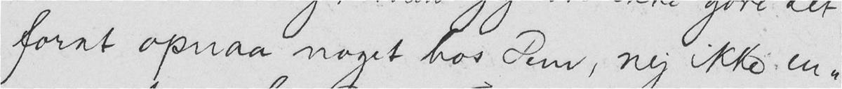forat opnaa noget hos Dem, nej ikke en-
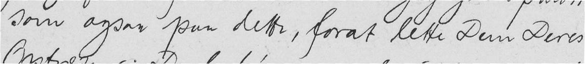som ogsaa paa dette, forat lette Dem Deres
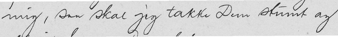mig, saa skal jeg takke Dem stumt og
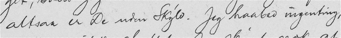altsaa er De uden Skyld. Jeg haabed ingenting,
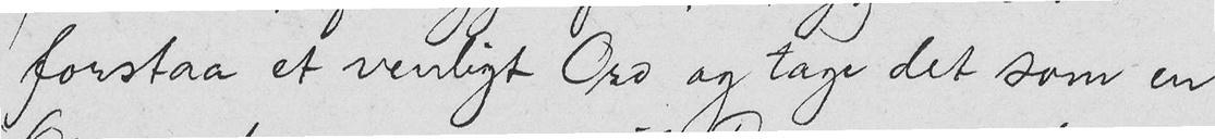forstaa et venligt Ord og tage det som en
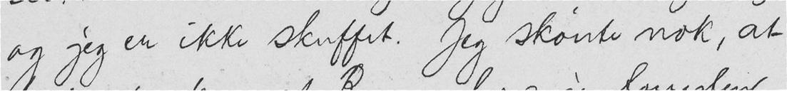og jeg er ikke skuffet. Jeg skønte nok, at
